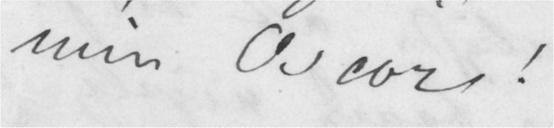min Oscar!
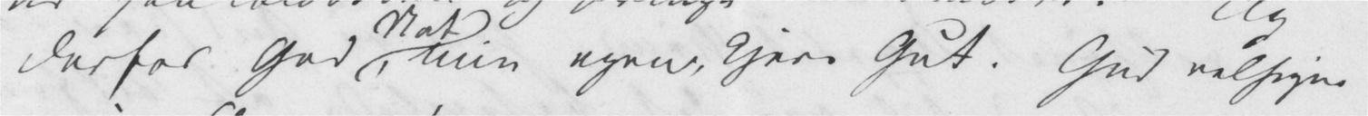derfor God Nat, min egen, kjere Gut. Gud velsigne
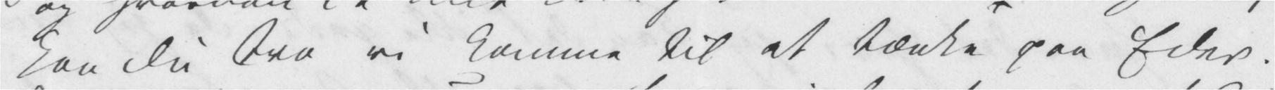kan Du tro vi komme til at tænke paa Eder.
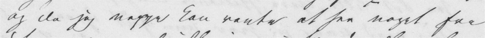og da jeg neppe kan vente at see noget fra
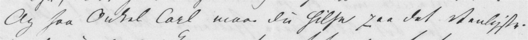Og saa Onkel Carl maa Du hilse paa det Venligste
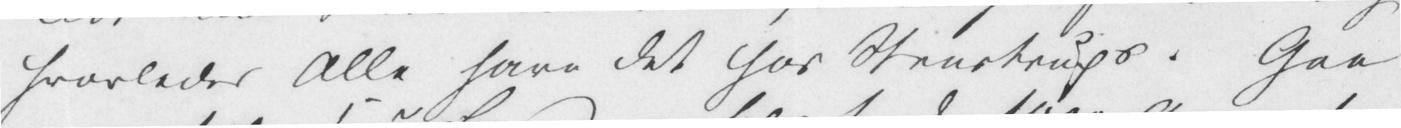hvorledes Alle have det hos Stenstrups. Gaa
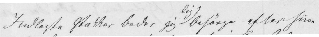Indlagte Pakker beder jeg Dig besørge efter sine
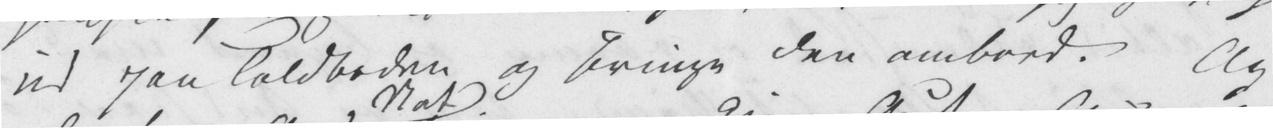ud paa Toldboden og bringe den ombord. Og
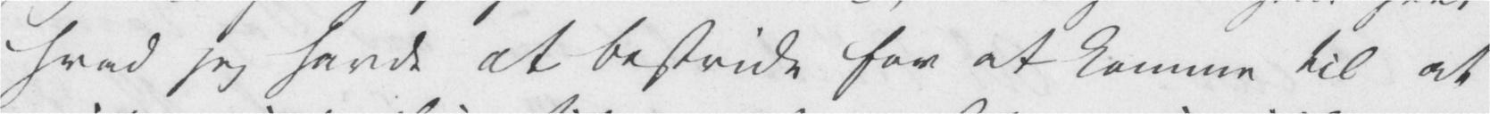hvad jeg havde at bestride for at komme til at
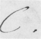C.
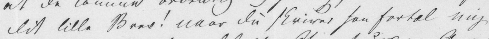Dit lille Brev! naar Du skriver saa fortæl mig
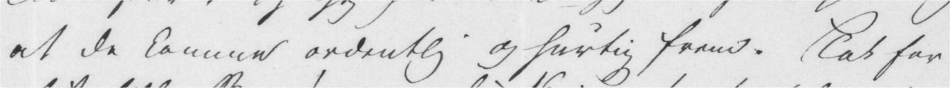at de komme ordentlig og hurtig frem. Tak for
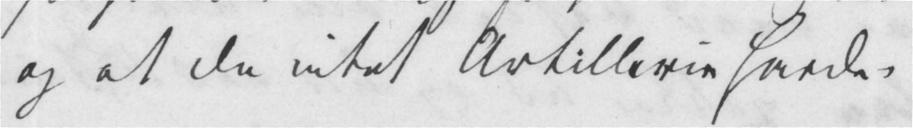og at Du intet Artillerie havde.
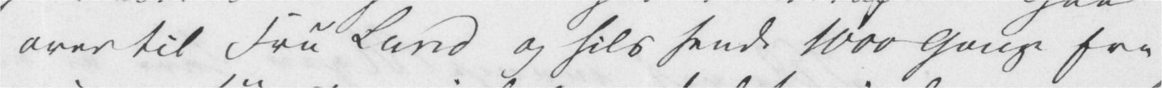over til Fru Lund og hils hende 1000 Gange fra
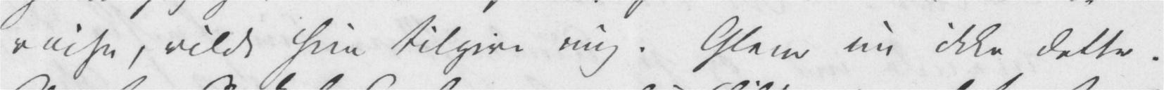reise, vilde hun tilgive mig. Glem nu ikke dette.
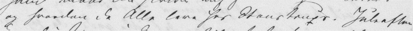og hvordan de Alle leve hos Stenstrups. Juleaften
